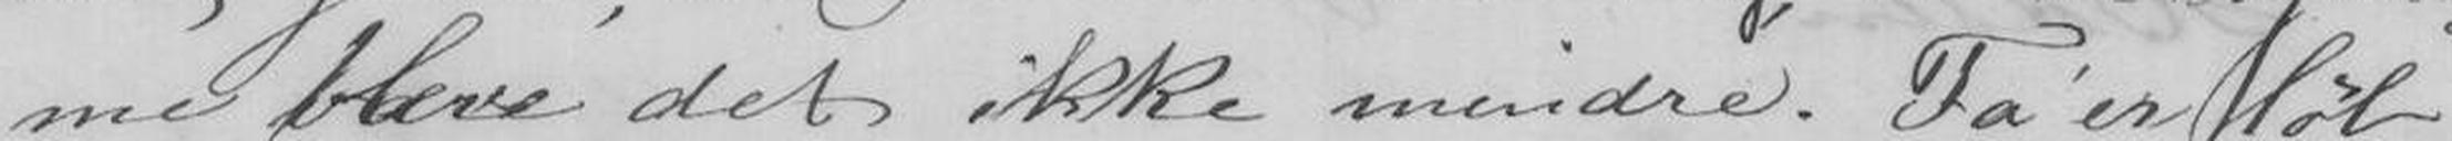me blive det ikke mindre. Fa'er løb
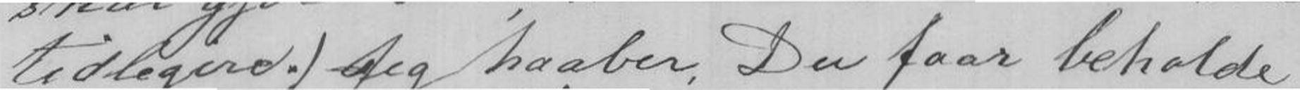tidligere.) Jeg haaber, Du faar beholde
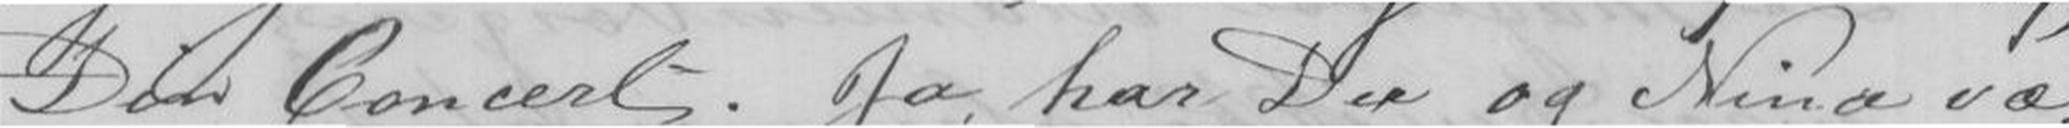Din Concert. Ja, har Du og Nina væ-
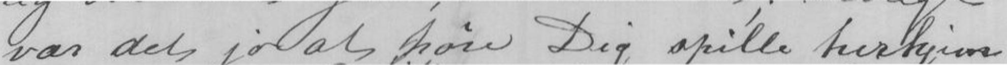var det jo at høre Dig spille herhjem-
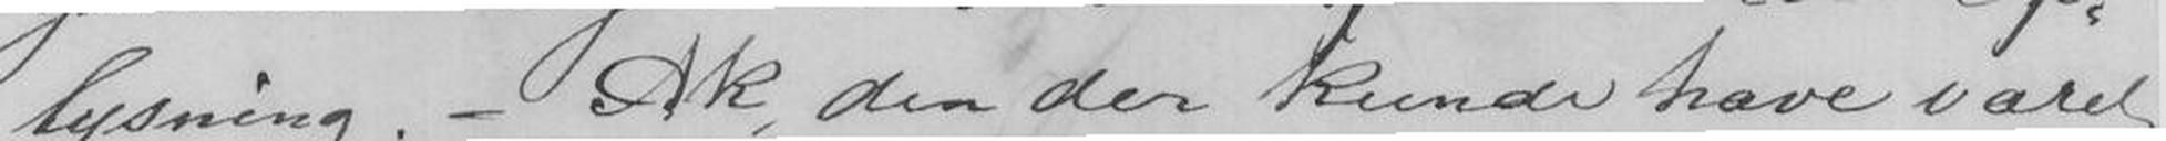lysning. - Ak, den der kunde have været
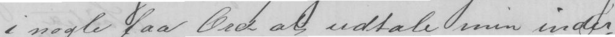i nogle faa Ord at udtale min inder-
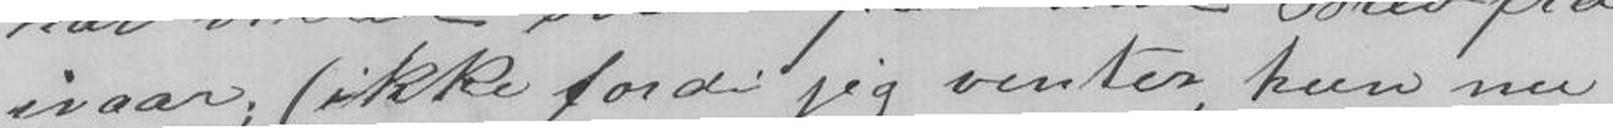ivaar; (ikke fordi jeg venter, hun nu
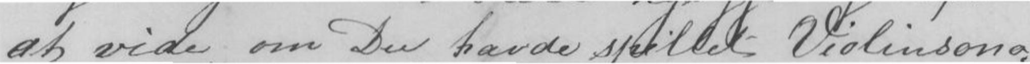at vide, om Du havde spillet Violinsona-
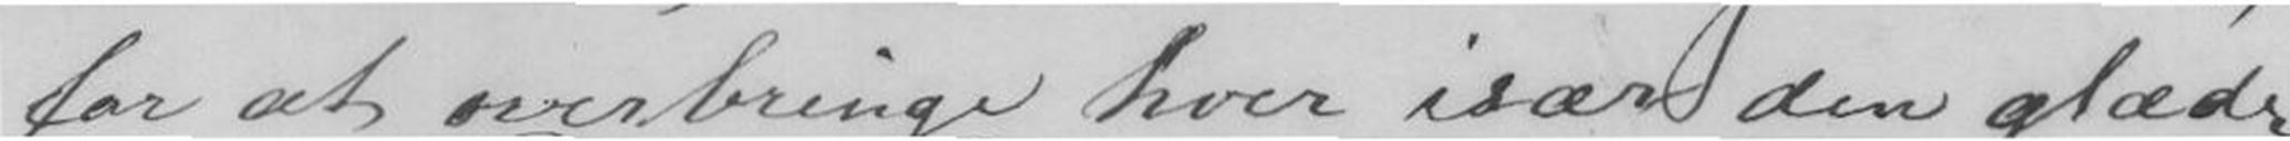for at overbringe hver især den glæde-
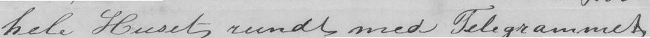hele Huset rundt med Telegrammet,
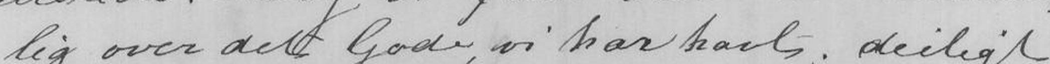lig over det Gode, vi har havt; deiligt
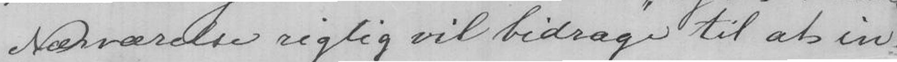Nærværelse rigtig vil bidrage til at in-
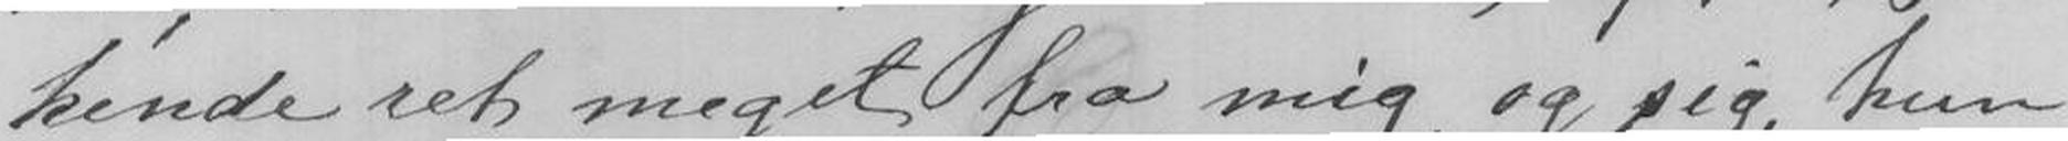hende ret meget fra mig og sig, hun
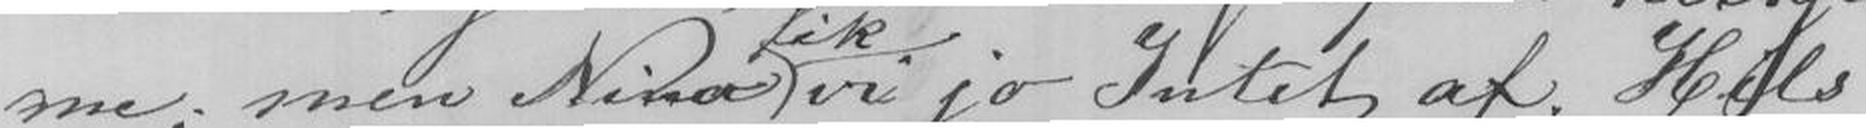me; men Nina vi jo Intet af. Hils
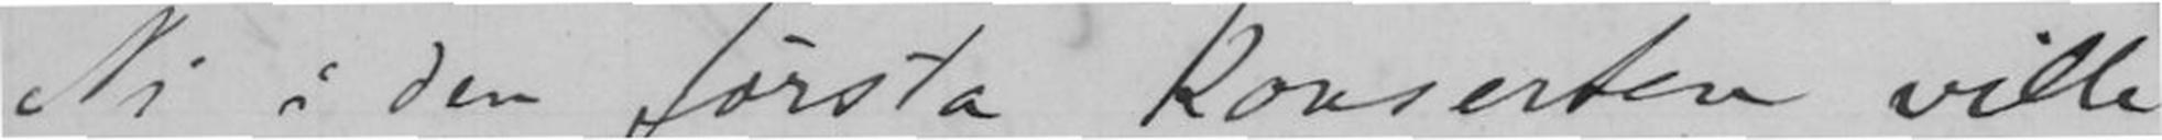Ni i den första konserten ville
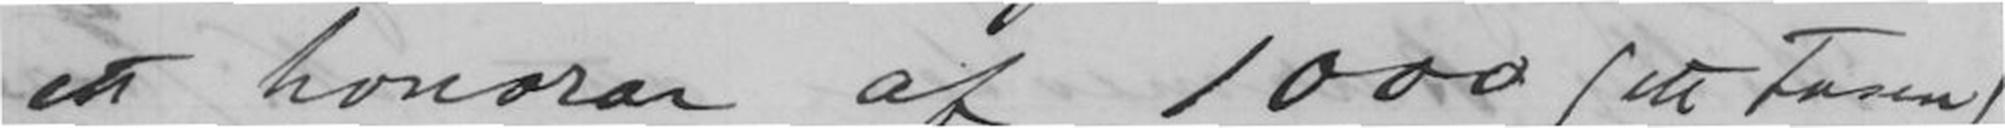ett honorar af 1000 (ett Tusen)
